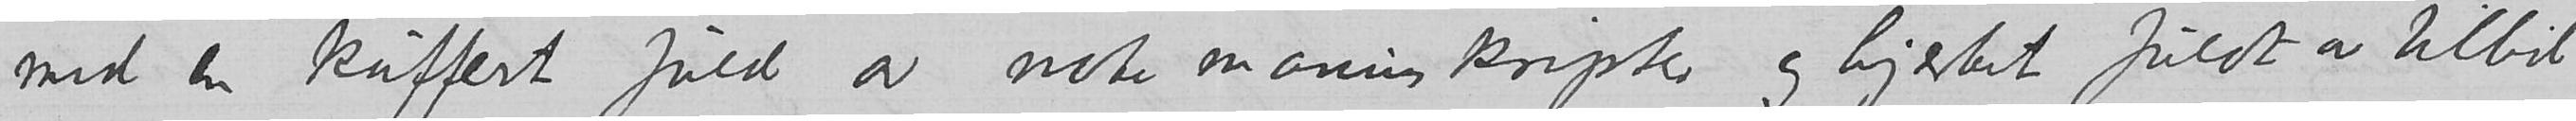med en kuffert fuldt av note manuskripter og hjertet fuldt av tillit
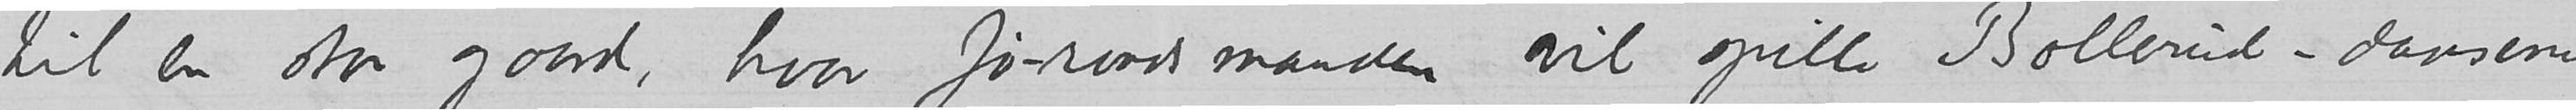til en stor gaard, hvor jø-raadsmanden vil spille Bollerud-dansen
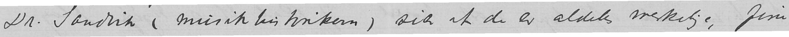Dr. Sandvik (musikhistorikeren) sier at de er aldeles merkelige, fine,
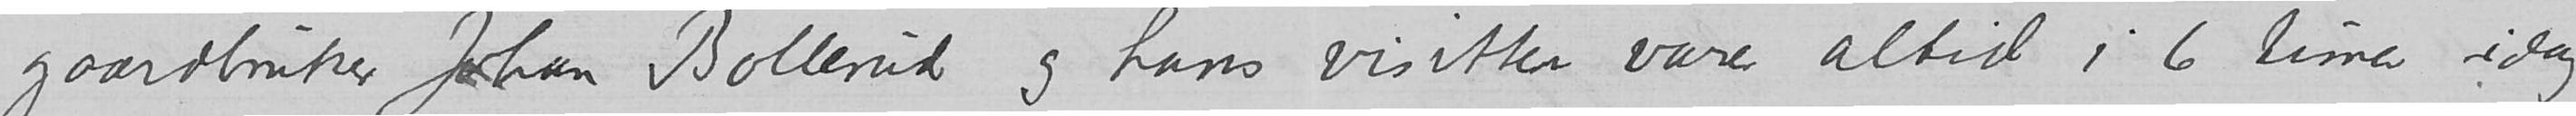gaardbruker Johan Bollerud og hans visitter varer altid i 6 timer idag
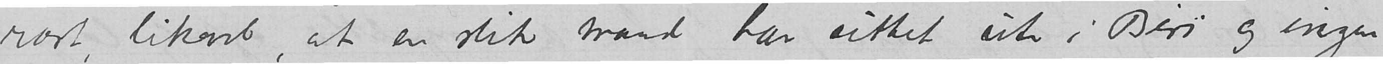rart, likevel, at en slik mand har sittet ute i Biri og ingen
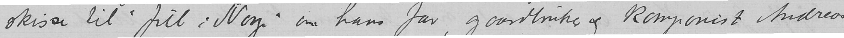skisse til «Jul i Norge» om hans far, gaardbruker og komponist Andreas
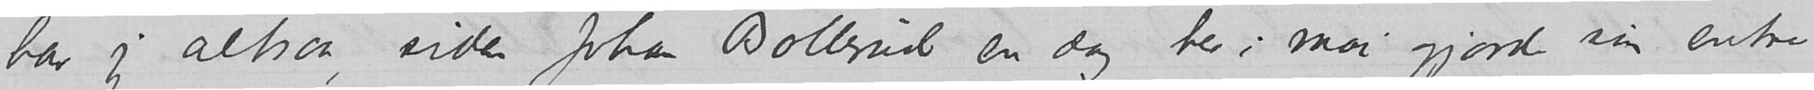har jeg altsaa, siden Johan Bollerud en dag her i mai gjorde sin entre
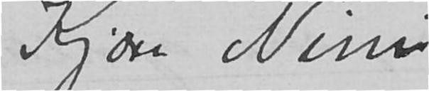Kjære Nini
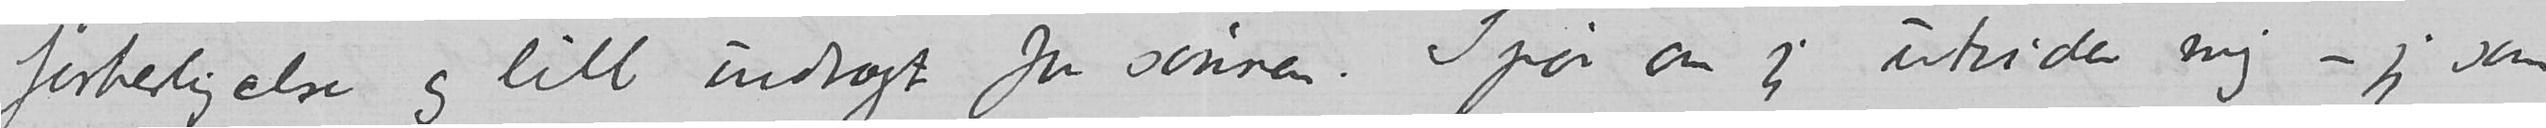forherligelse og litt indtægt for sønnen. Spør om jeg utvider mig – jeg som
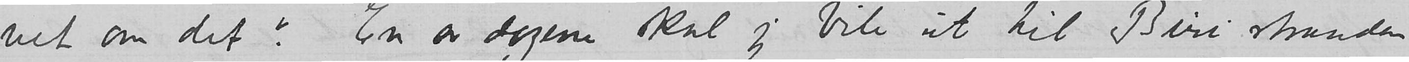vet om det? En av dagene skal jeg bile ut til Biri stranden
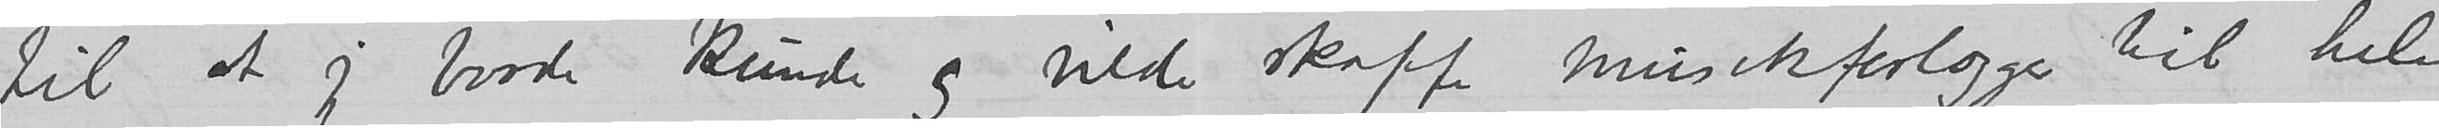til at jeg burde kunde og vilde skaffe musikforlegger til hele
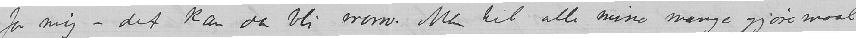for mig – det kan da bli morro. Men til alle mine mange gjøremaal
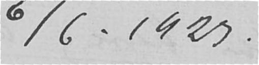6/6.1923
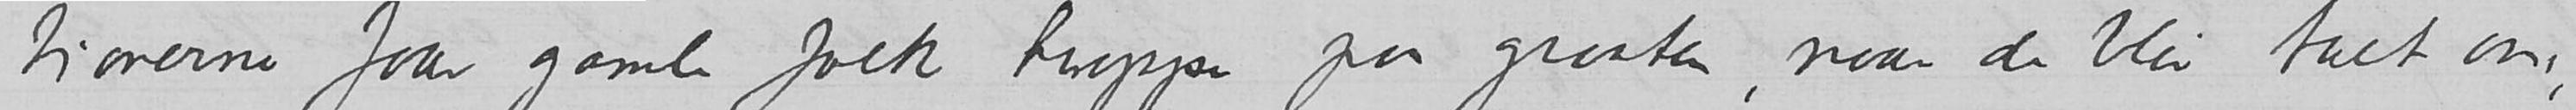tionerne faar gamle folk heroppe paa graaten, naar de blir talt om, og
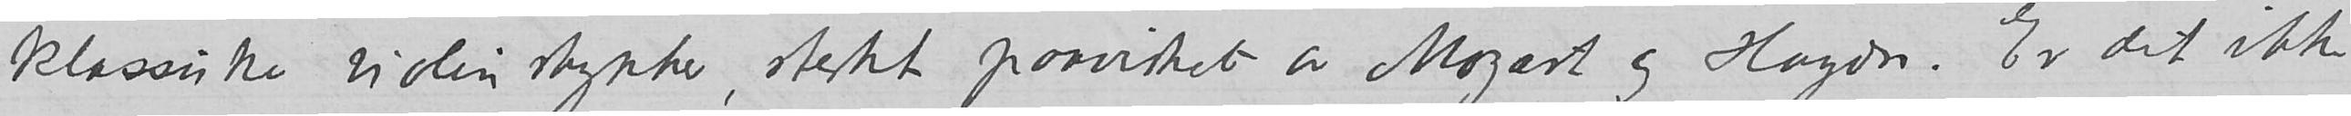klassiske violinstykker, sterkt paavirket av Mozart og Haydn. Er det ikke
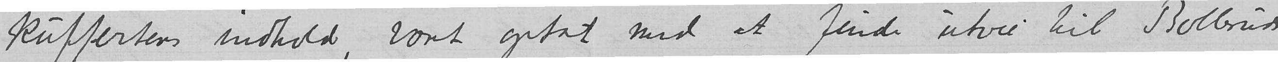kuffertens indhold, været optat med at finde utvei til Bolleruds
